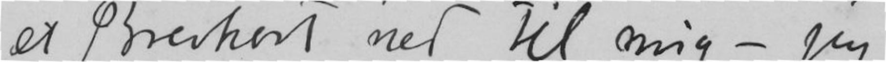et Brevkort ned til mig - jeg
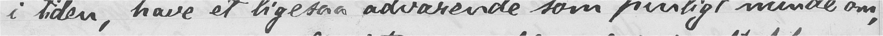i tiden, have et ligesaa advarende som pinligt minde om,
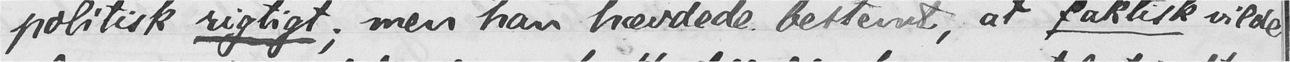politisk rigtigt; men han hævdede bestemt, at faktisk vilde
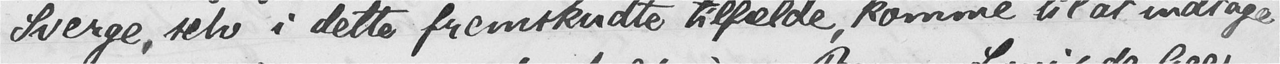Sverge selv i dette fremskudte tilfælde, komme til at indtage
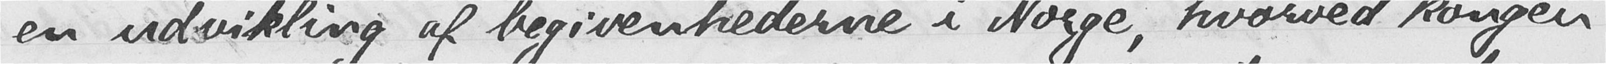en udvikling af begivenhederne i Norge, hvorved kongen
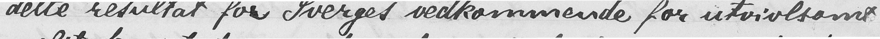dette resultat for Sverges vedkommende for utvivlsomt
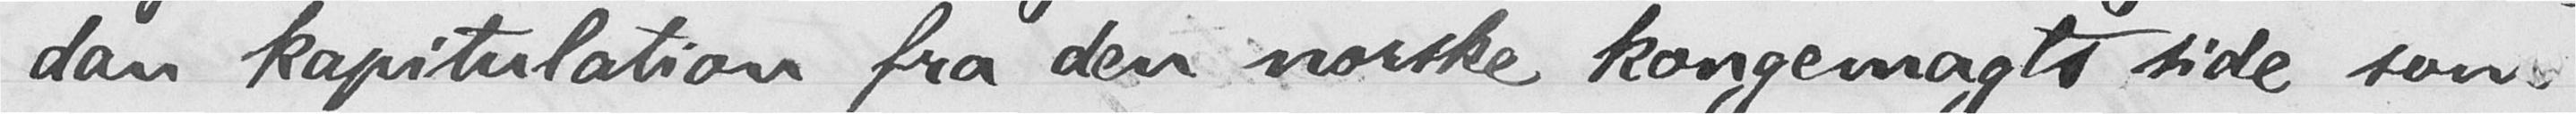dan kapitulation fra den norske kongemagts side som
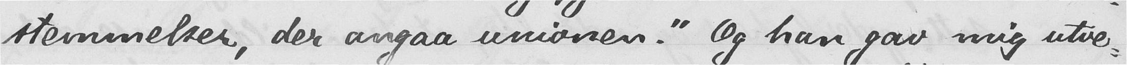stemmelser, der angaa unionen." Og han gav mig utve-
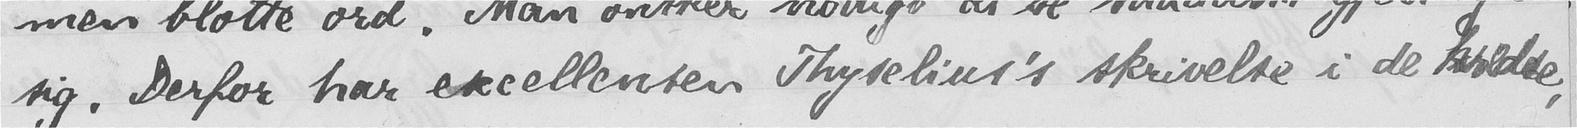sig. Derfor har excellensen Thyselius's skrivelse i de kredse,
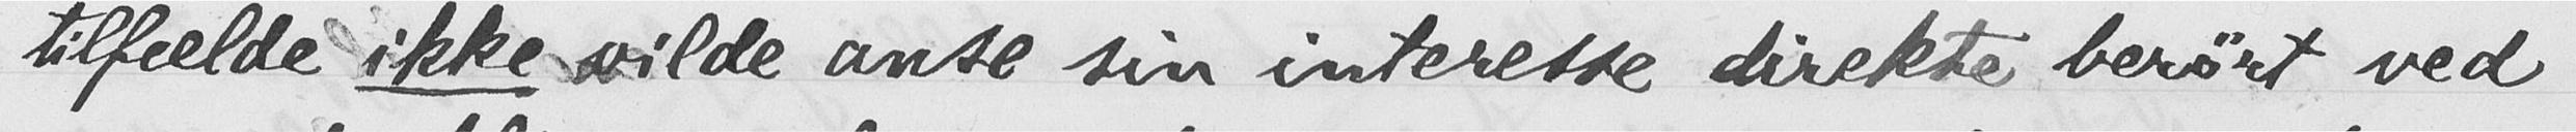tilfælde ikke vilde anse sin interesse direkte berørt ved
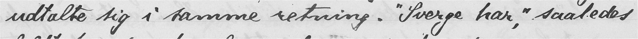udtalte sig i samme retning. "Sverge har", saaledes
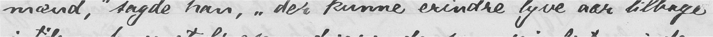mænd," sagde han, "der kunne erindre tyve aar tilbage
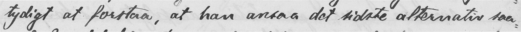tydigt at forstaa, at han ansaa det sidste alternativ saa-
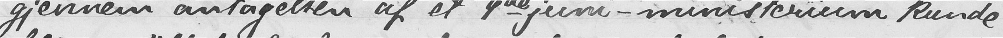gjennem antagelsen af et 9de juni-ministerium kunde
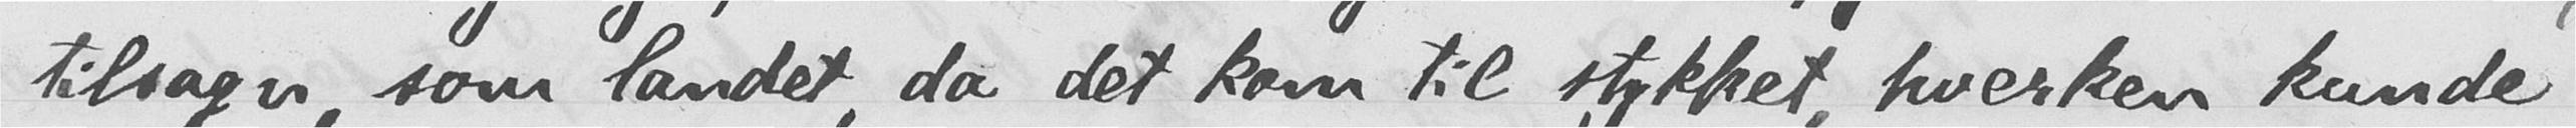tilsagn, som landet, da det kom til stykket, hverken kunde
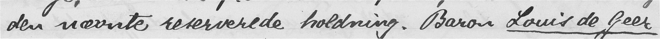den nævnte reserverede holdning. Baron Louis de Geer
